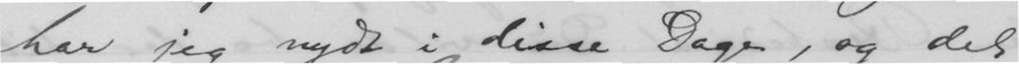har jeg nydt i disse Dage, og det
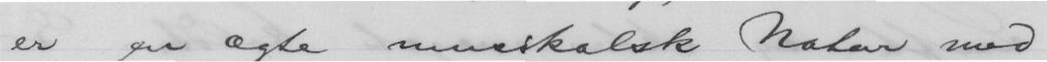er en ægte musikalsk Natur med
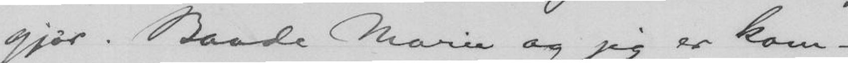gjør. Baade Marie og jeg er kom-
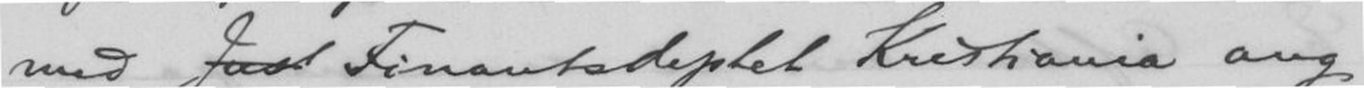med Just Finantsdeptet Kristiania ang.
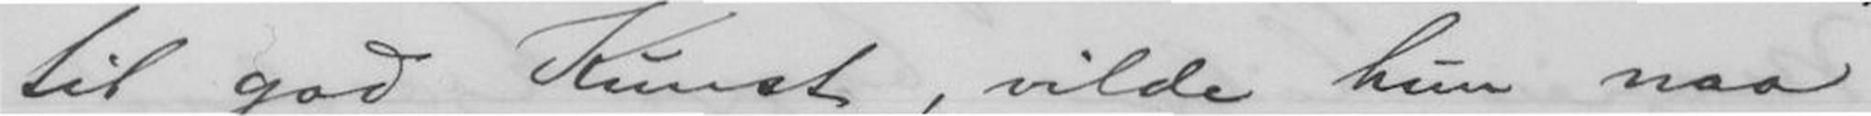til god Kunst, vilde hun naa
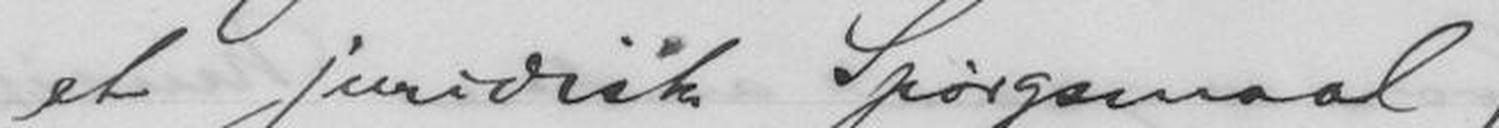et juridisk Spørgsmaal)
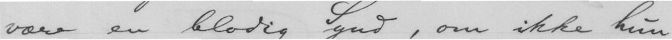være en blodig Synd, om ikke hun
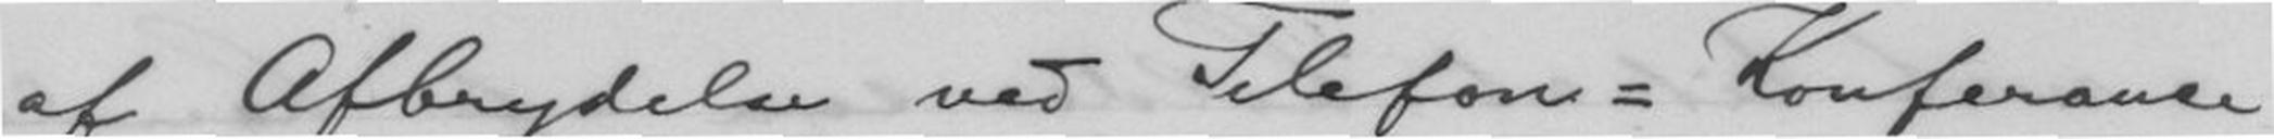af Afbrydelse ved Telefon=Konferance
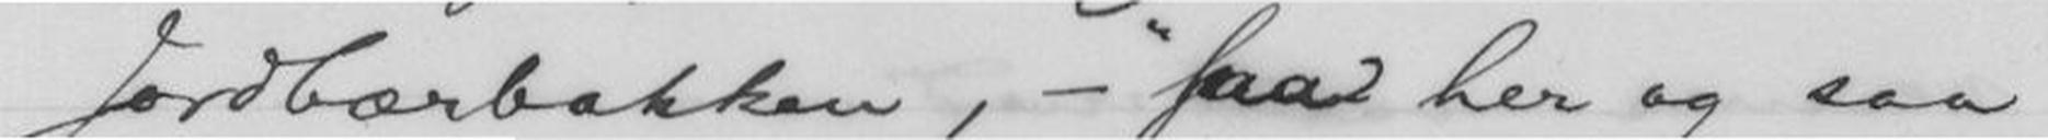Jordbærbakken, - "saa her og saa
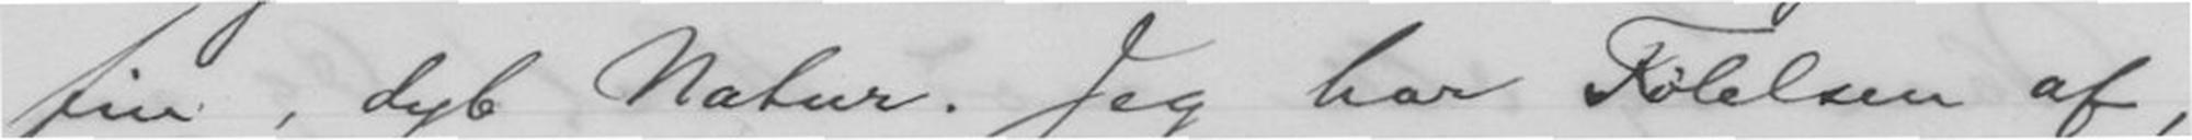fin, dyb Natur. Jeg har Følelsen af,
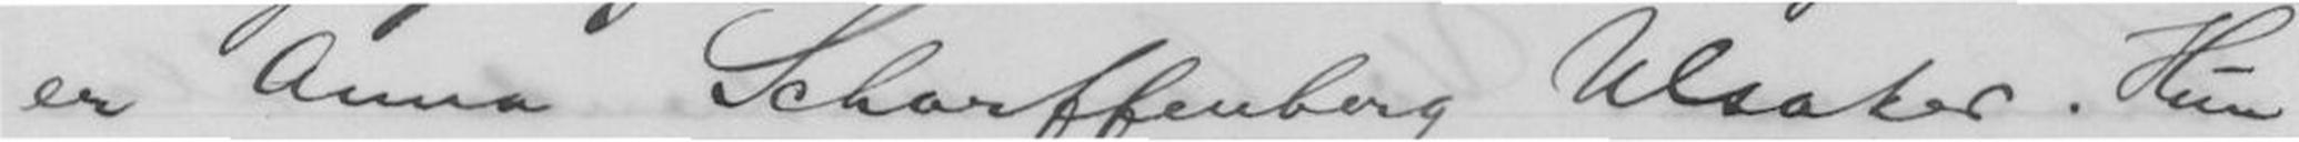er Anna Scharffenberg Ulsaker. Hun
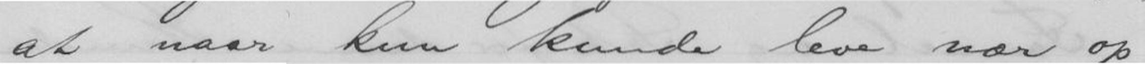at naar kun kunde leve nær op
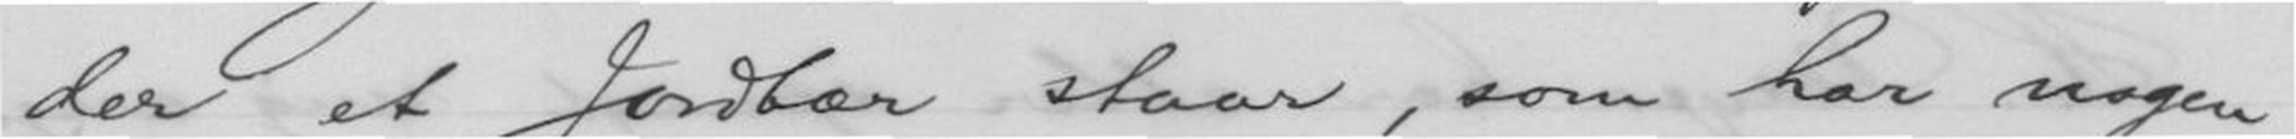der et Jordbær staar, som har nogen
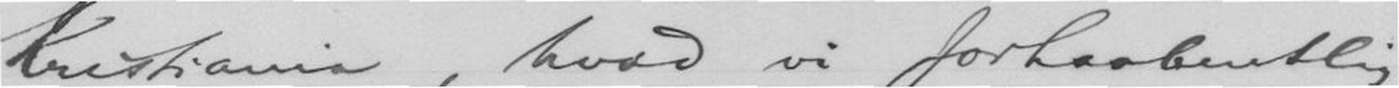Kristiania, hvad vi forhaabentlig
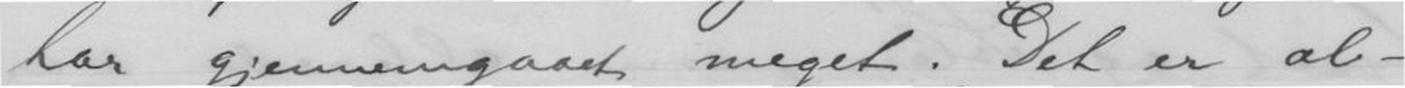har gjennemgaaet meget. Det er al-
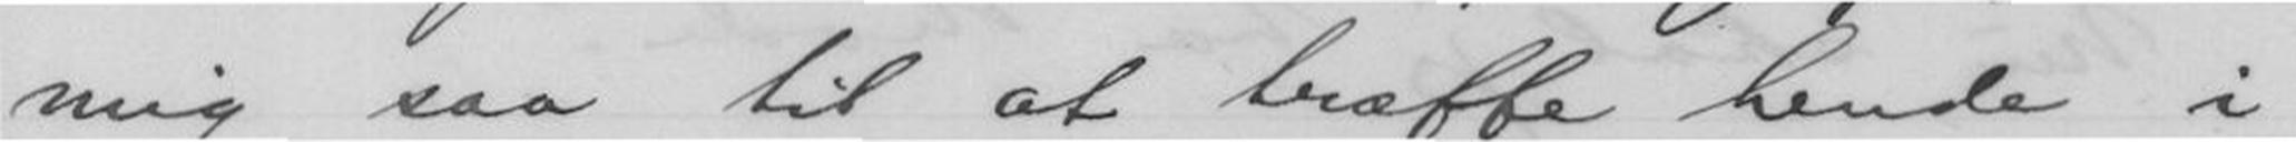mig saa til at træffe hende i
